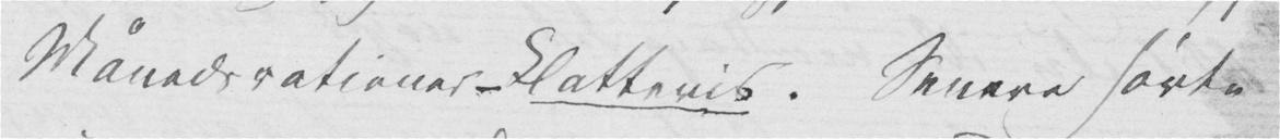Månedsrationer – Klattevis. Senere hørte
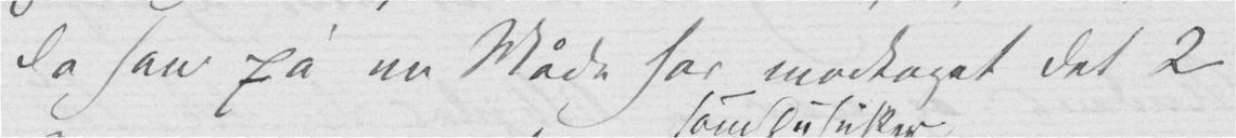da han på en Måde har modtaget det 2
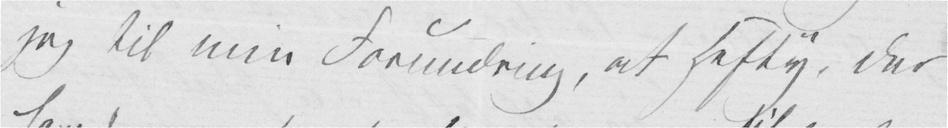jeg til min Forundring, at Hefty, der
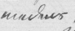medens
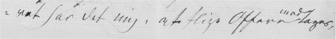ret har det mig, at slige Offere tages,
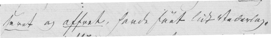seret og offret, havde fået lidt Vederlag,
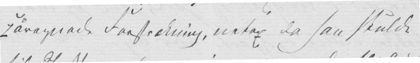påregnede Forstrækning, netop da han skulde
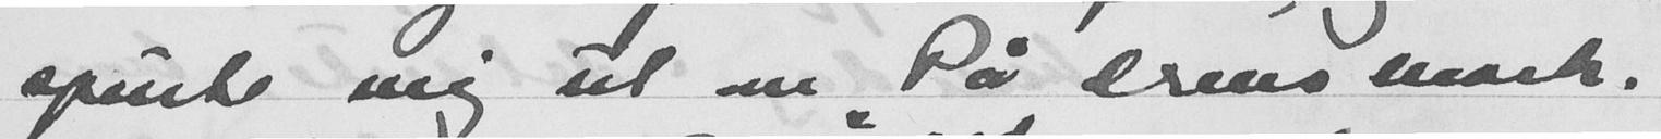spurte mig ut om "På ærens mark."
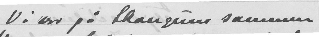Vi var på Skaugum sammen
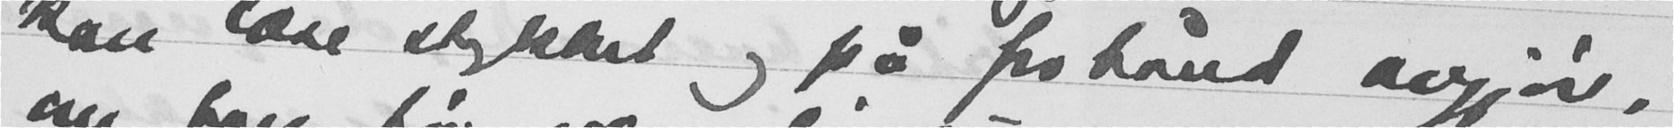kan lese stykket og på forhånd avgjøre,
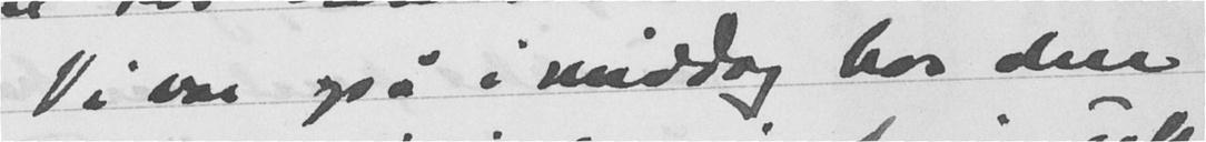Vi var også i middag hos den
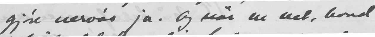gjøre nervøs ja. Og når en vet, hvad
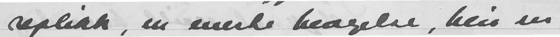replikk, en eneste bevegelse, blir en
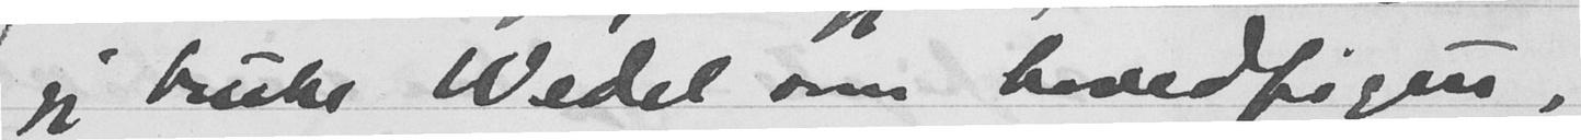jeg bruke Wedel som hovedfigur,
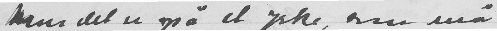men det er også et yrke, som må
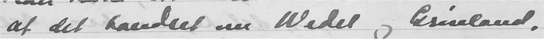at det handlet om Wedel og Øverland,
